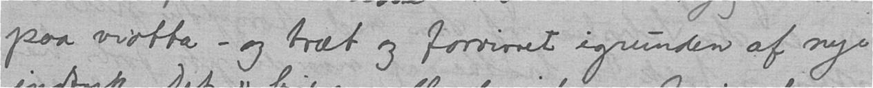paa viotta - og træt og forvirret igrunden af nye
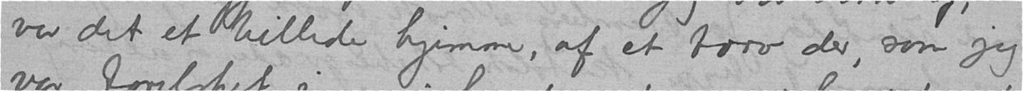var det et billede hjemme, af et torv der, som jeg
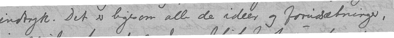indtryk. Det er ligesom alle de ideer og forudsætninger,
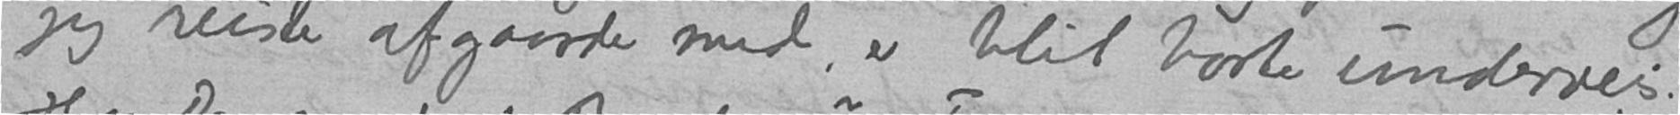jeg reiste afgaarde med er blit borte underveis. -
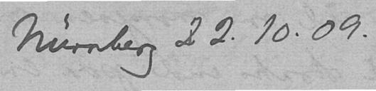Nürnberg 22.10.09.
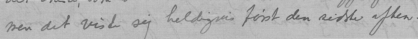men det viste sig heldigvis først den sidste aften.
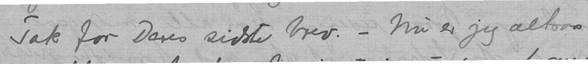Tak for Deres sidste brev. - Nu er jeg altsaa
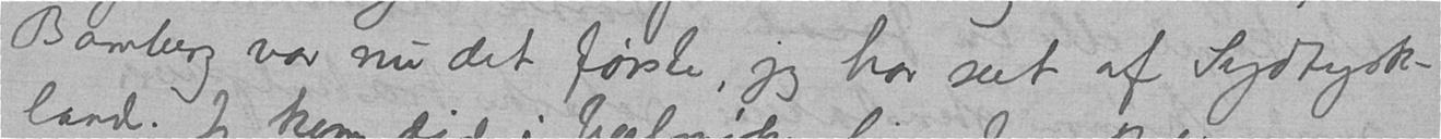Bamberg var nu det første, jeg har seet af Sydtysk-
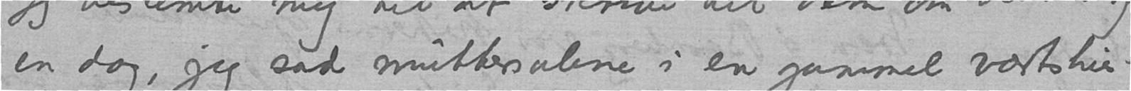en dag, jeg sad muttersalene i en gammel værtshus-
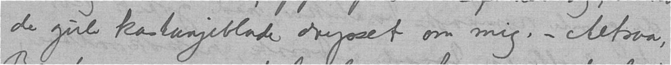de gule kastanjeblade drysset om mig. - Altsaa,
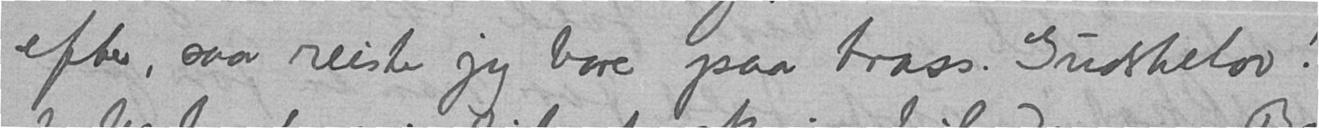efter, saa reiste jeg bare paa trass. Gudskelov!
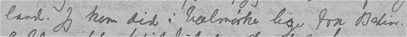land. Jeg kom did i bælmørke lige fra Berlin.
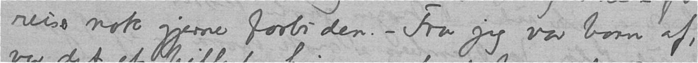reiser nok gjerne forbi den. - Fra jeg var barn af,
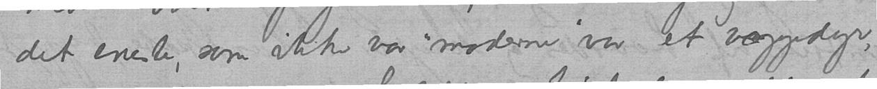det eneste, som ikke var "moderne" var et væggedyr,
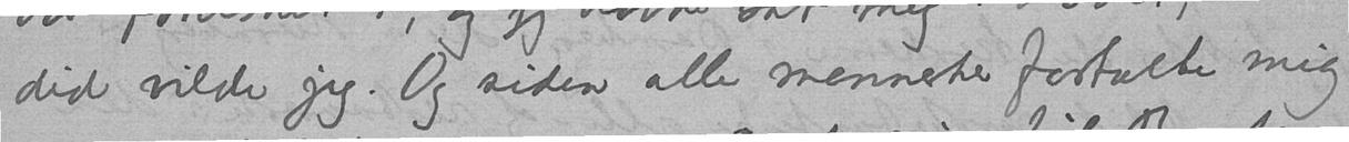dit vilde jeg. Og siden alle mennesker fortalte mig,
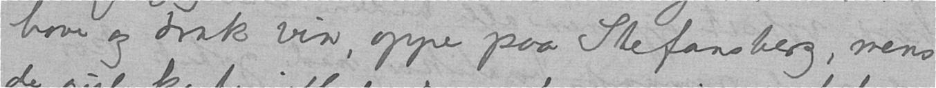have og drak vin, oppe paa Stefansberg, mens
